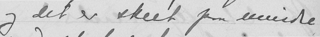og det er skeet paa mindre
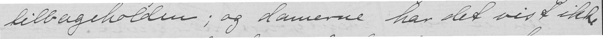tilbageholden; og damerne har det vist ikke
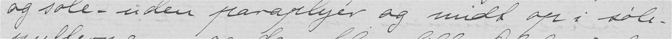og søle – uden paraplyer og midt op i søle-
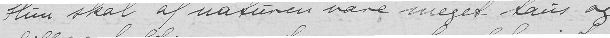Hun skal af naturen være meget taus og
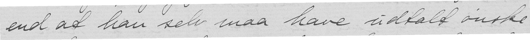end at han selv maa have udtalt ønske
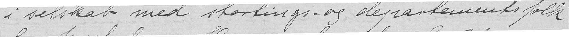i selskab med stortings- og departementsfolk
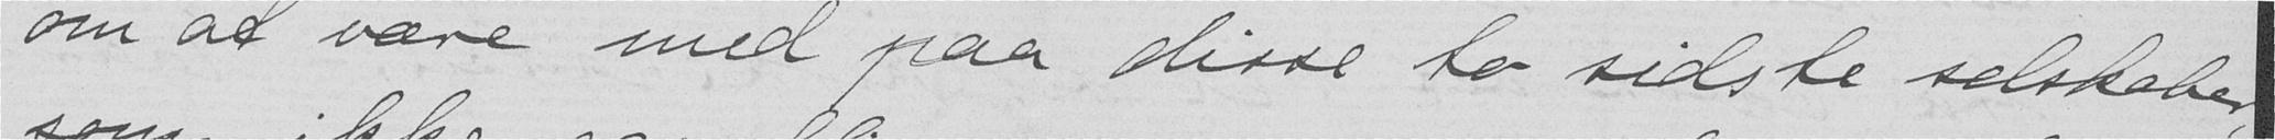om at være med paa disse to sidste selskaber,
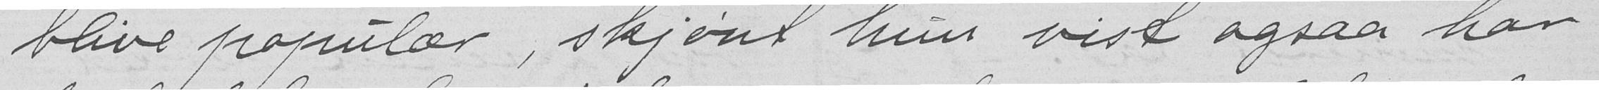blive populær, skjønt hun vist ogsaa har
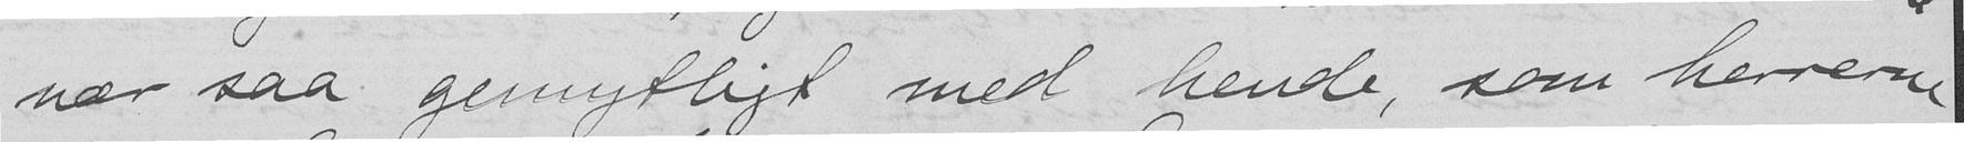nær saa gemytligt med hende, som herrerne
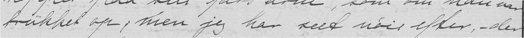trukket op; men jeg har seet nøie efter, – der
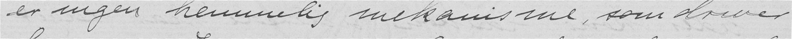er ingen hemmelig mekanisme, som driver
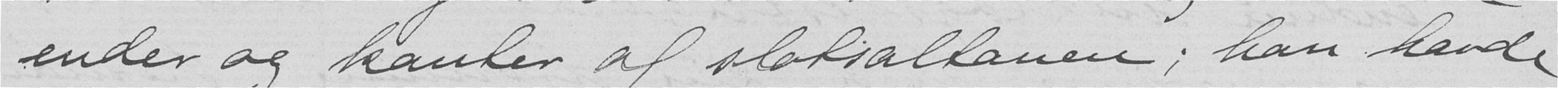ender og kanter af slotsaltanen; han havde
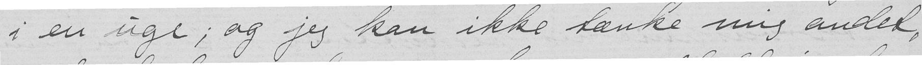i en uge; og jeg kan ikke tænke mig andet,
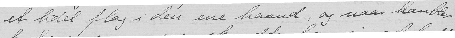et lidet flag i den ene haand, og naar han blev
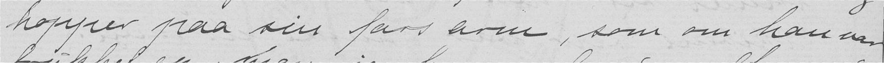hopper paa sin fars arm, som om han var
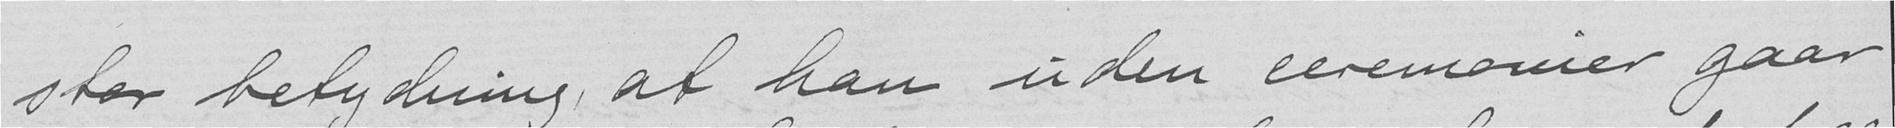stor betydning, at han uden ceremonier gaar
